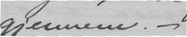gjennem. -
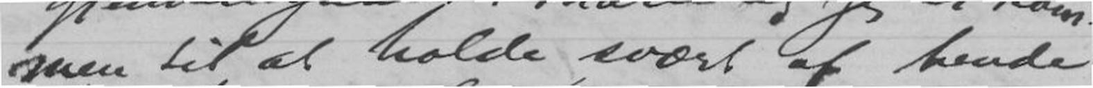men til at holde svært af hende
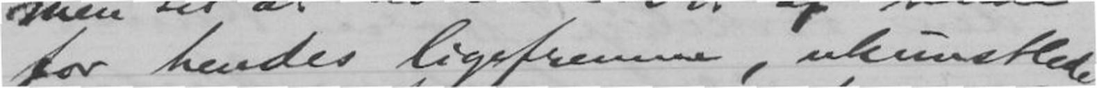for hendes ligefremme, ukunstlede
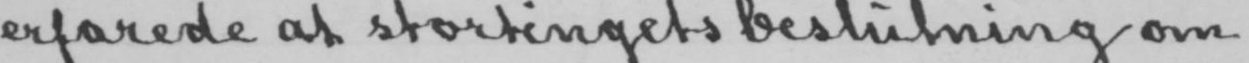erfarede at stortingets beslutning om
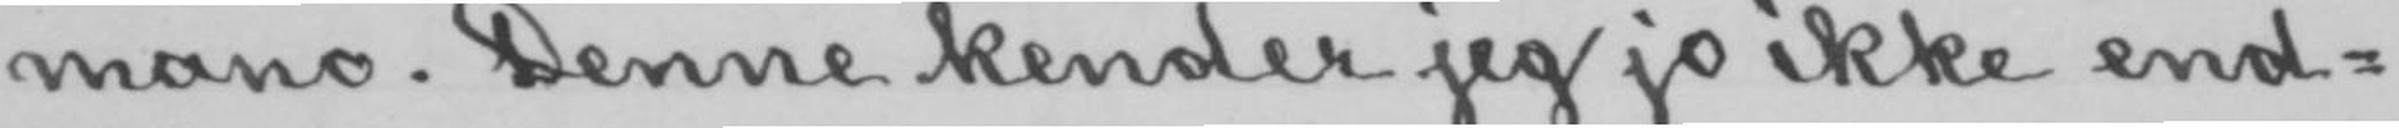mano. Denne kender jeg jo ikke end-
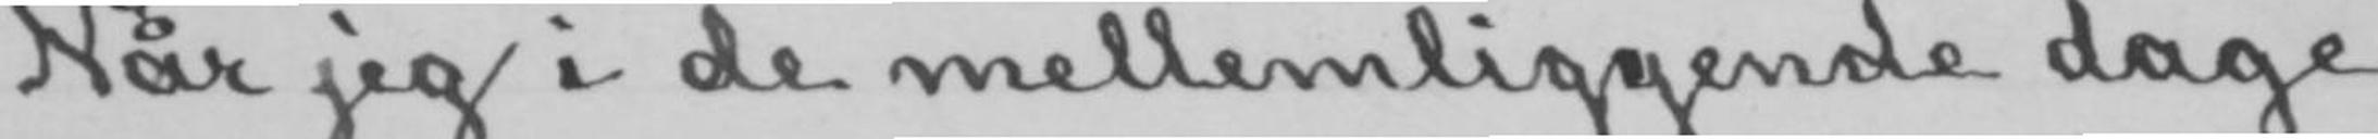Når jeg i de mellemliggende dage
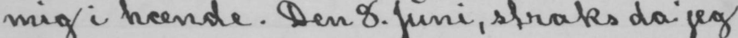mig i hænde. Den 8. Juni, straks da jeg
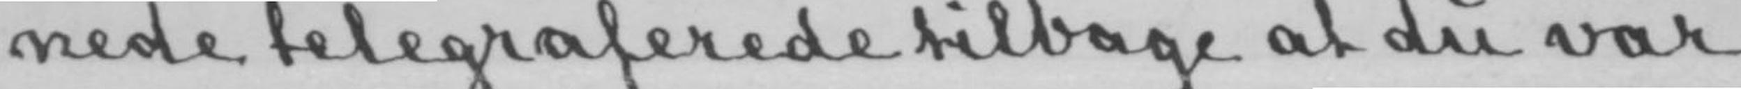nede telegraferede tilbage at du var
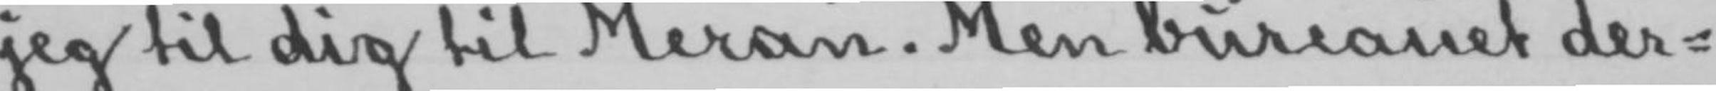jeg til dig til Meran. Men bureauet der-
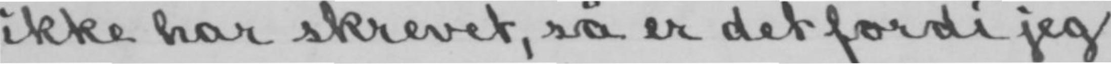ikke har skrevet, så er det fordi jeg
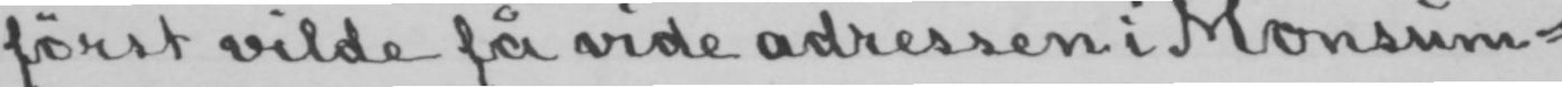først vilde få vide adressen i Monsum-
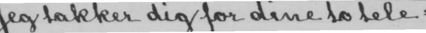Jeg takker dig for dine to tele-
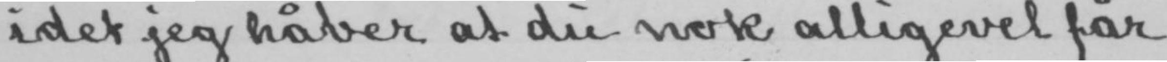idet jeg håber at du nok alligevel får
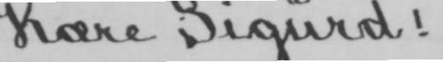Kære Sigurd!
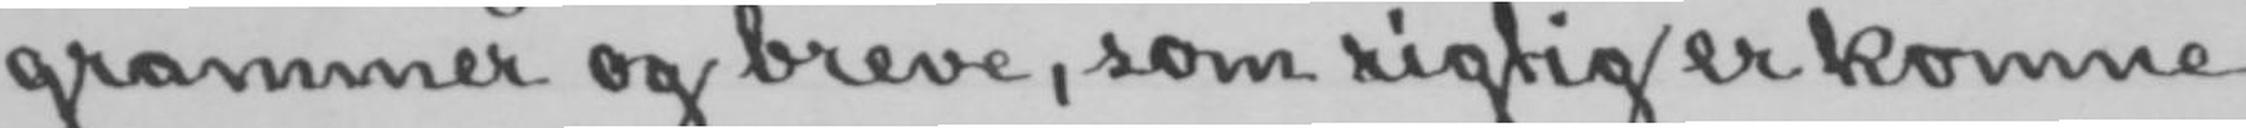grammer og breve, som rigtig er komne
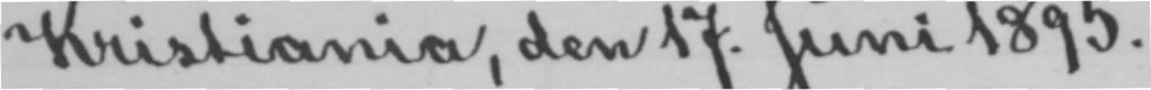Kristiania, den 17. Juni 1895.
